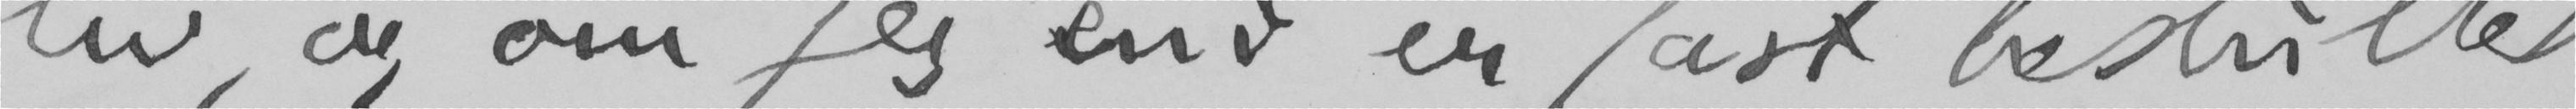liv, og om jeg end er fast besluttet
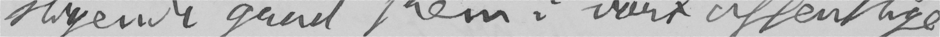stigende grad frem i vort offentlige
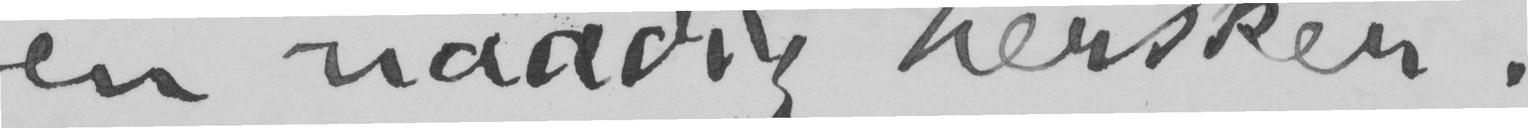en naadig hersker!
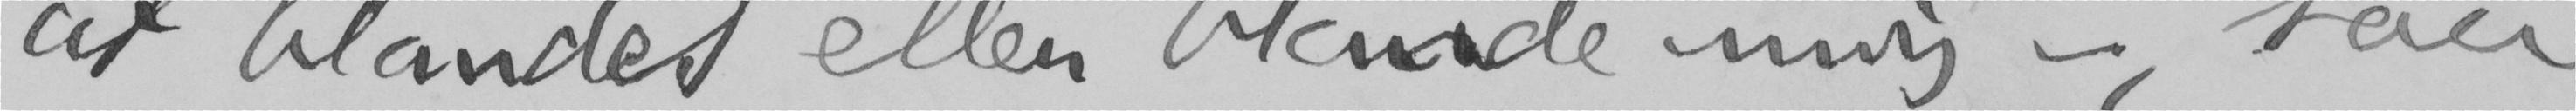at blandes eller blande mig –, saa
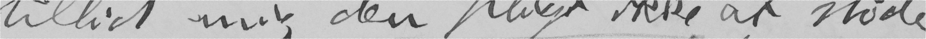tillid mig den pligt ikke at støde
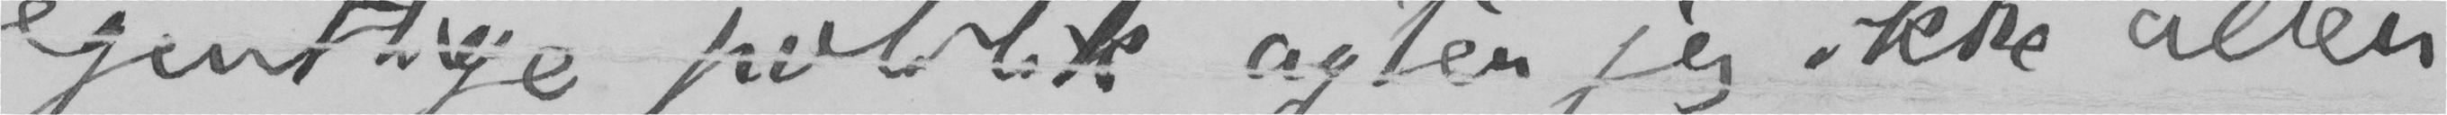egentlige politik agter jeg ikke atter
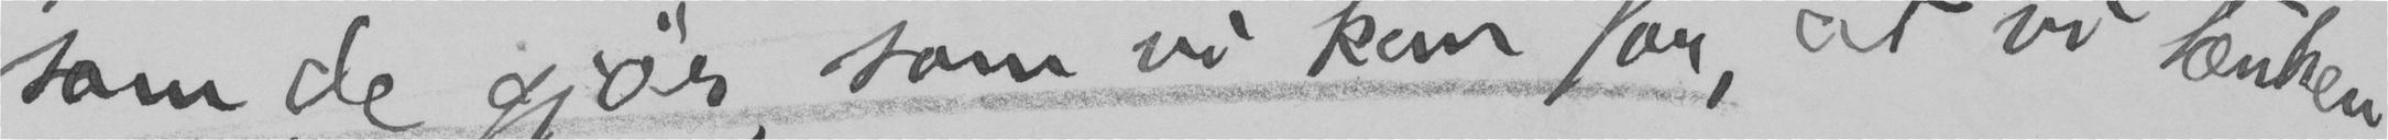som de gjør, som vi kan for, at vi tænker
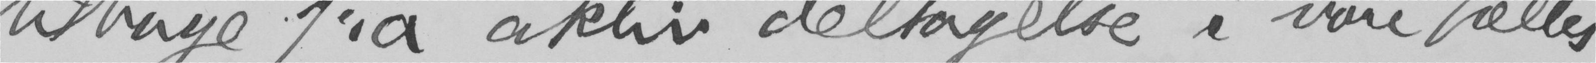tilbage fra aktiv deltagelse i vore fælles
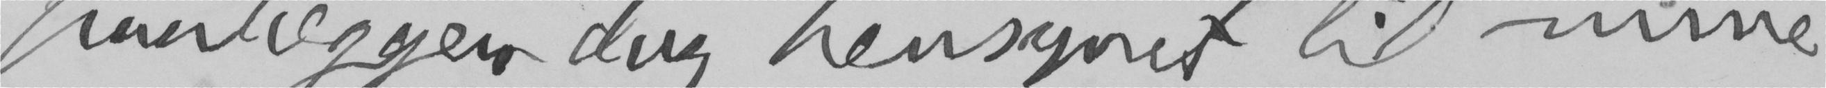paalægger dog hensynet til mine
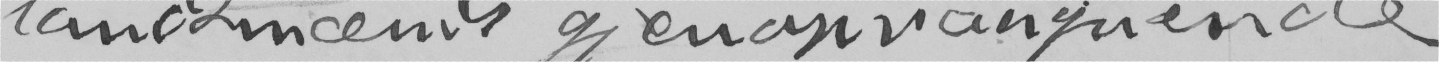landsmæds gjenopvaagnende
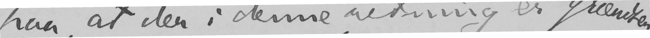paa, at der i denne retning er grændser,
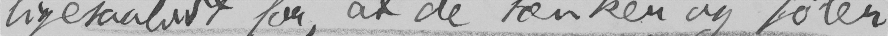ligesaalidt for, at de tænker og føler,
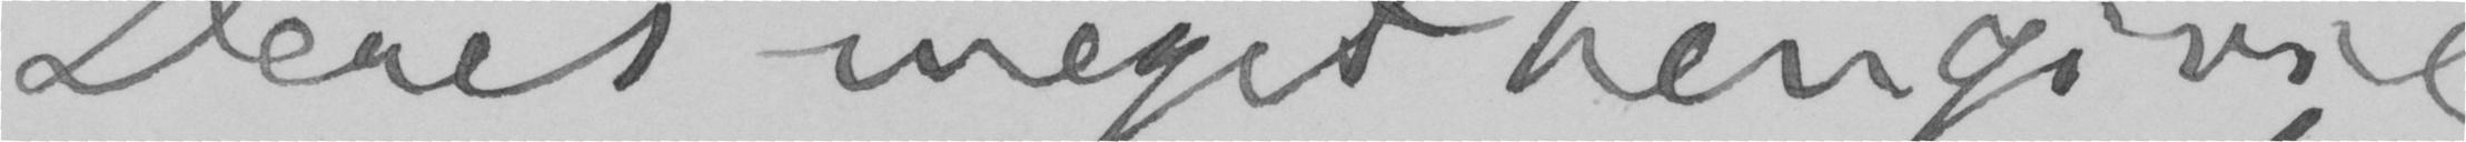Deres meget hengivne
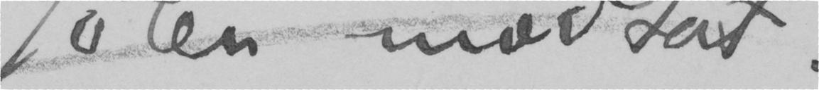og føler modsat.
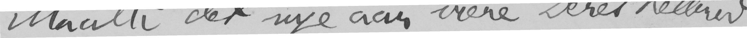Maatte det nye aar være Deres helbred
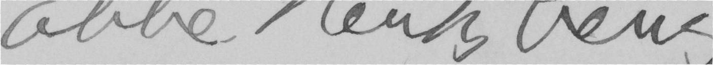Ebbe Hertzberg.
